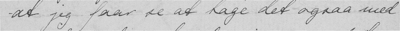at jeg faar se at tage det ogsaa med
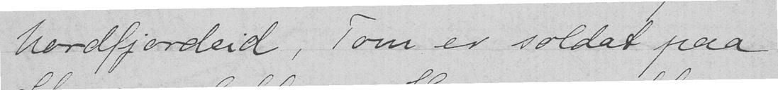Nordfjordeid, Tom er soldat paa
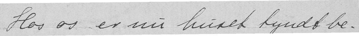Hos os er nu huset tyndt be-
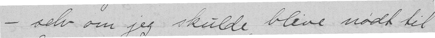- selv om jeg skulde blive nødt til
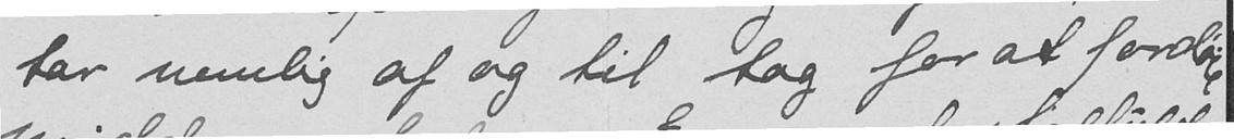tar nemlig af og til tag for at fordag
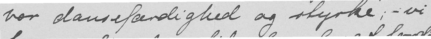vor dansefærdighed og styrke, - vi
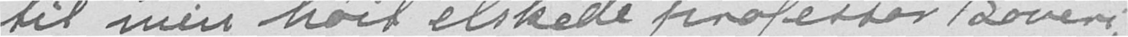til min høit elskede professor Boveri
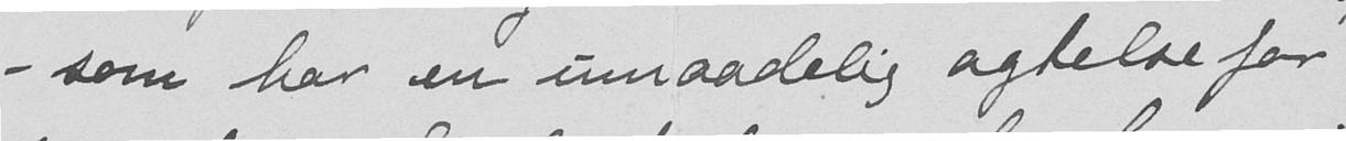- som har en umaadelig agtelse for
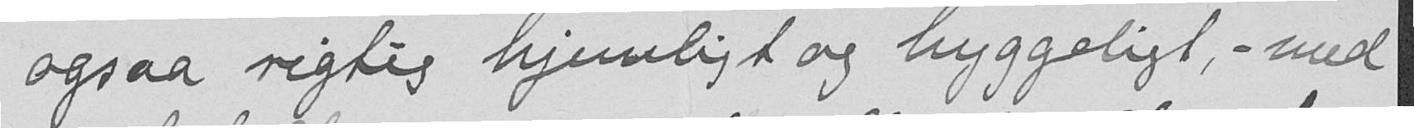ogsaa rigtig hjemligt og hyggeligt - med
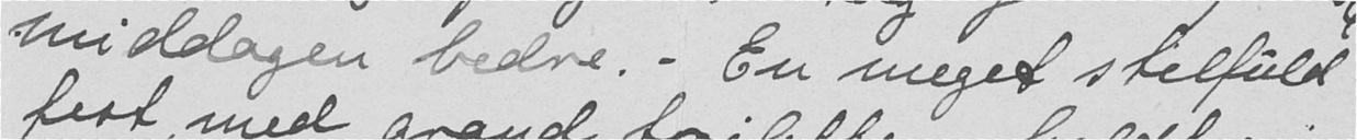middagen bedre, - En meget stilfuld
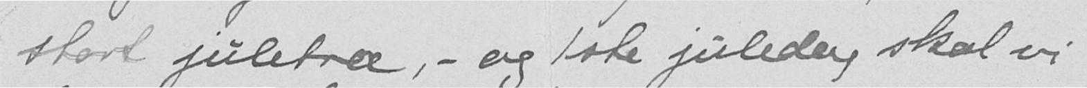stort juletræ,- og 1ste juledag skal vi
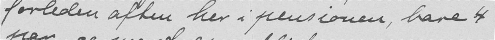forleden aften her i pensionen, bare 4
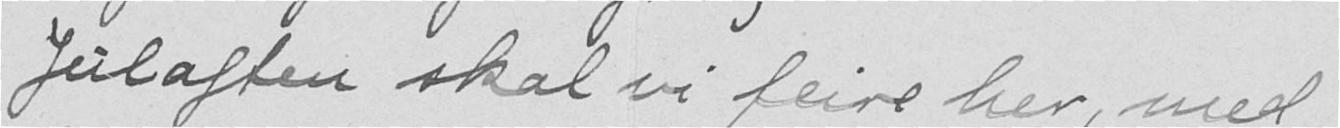Julaften skal vi feire her, med
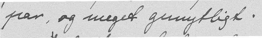par, og meget gemytligt.
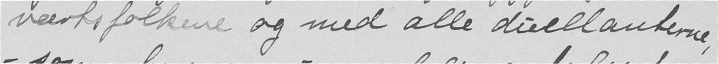værtsfolkene og med alle duellanterne
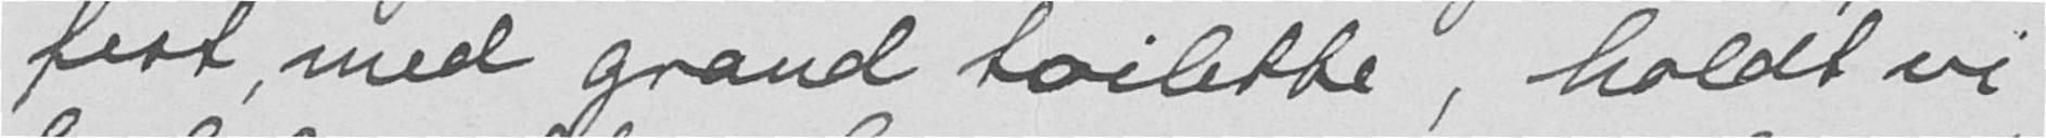fest med grand toilette, holdt vi
